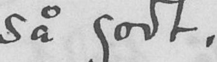så godt.
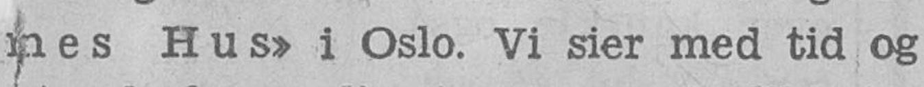nes Hus" i Oslo. Vi sier med tid og
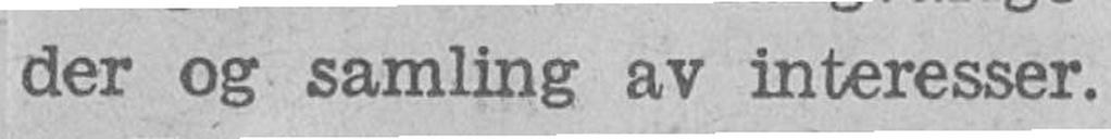der og samling av interesser.
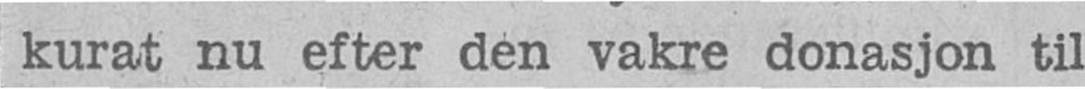kurat nu efter den vakre donasjon til
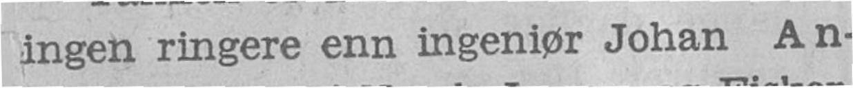ingen ringere enn ingeniør Johan An-
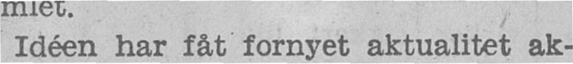Idéen har fåt fornyet aktualitet ak-
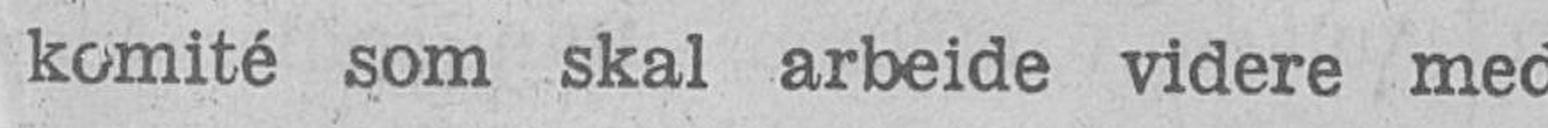komité som skal arbeide videre med
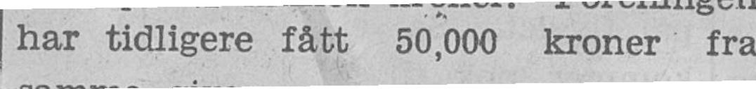har tidligere fått 50,000 kroner fra
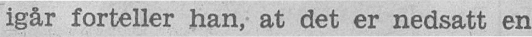igår forteller han, at det er nedsatt en
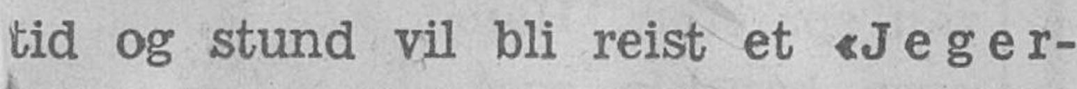tid og stund vil bli reist et "Jeger-
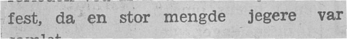fest, da en stor mengde jegere var
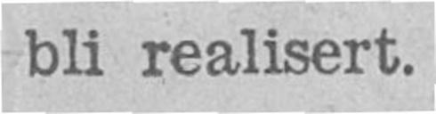bli realisert.
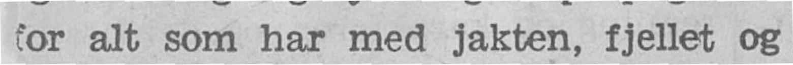for alt som har med jakten, fjellet og
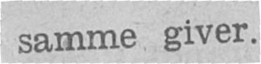samme giver.
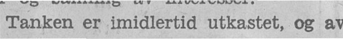Tanken er imidlertid utkastet, og av
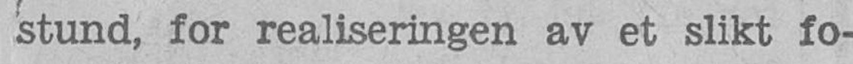stund, for realiseringen av et slikt fo-
